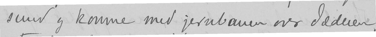sund og komme med jernbanen over Jæderen,
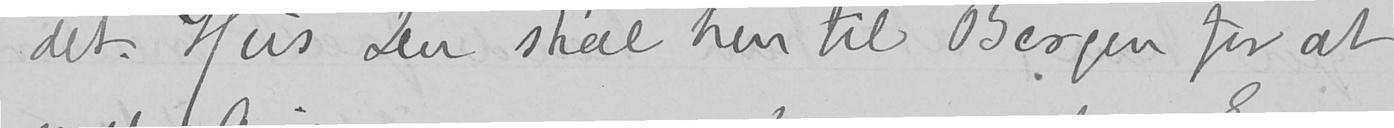det. Hvis Du skal hen til Bergen for at
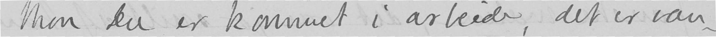Mon Du er kommet i arbeide, det er van-
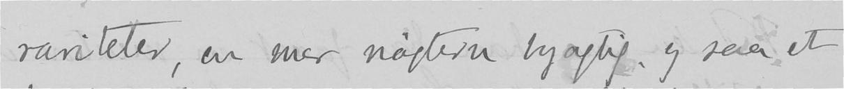rariteter, en mer nøgtern byagtig, og saa et
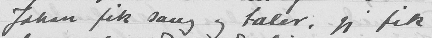Johan fik sang og taler, jeg fik
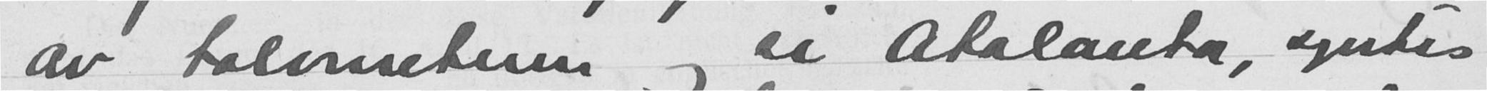av tolvmeteren og si Atalanta, syntes
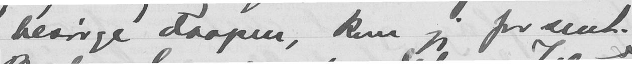besørge daapen, kom jeg for sent.
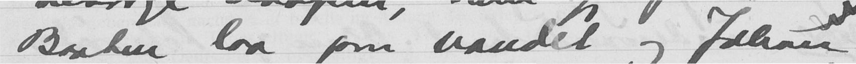Baaten laa paa vandet og Johan
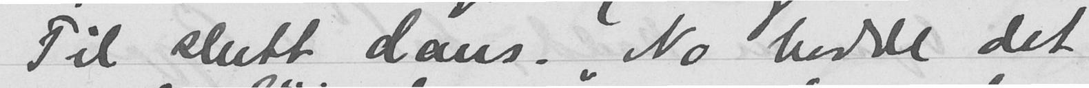Til slutt dans. "No hadde det
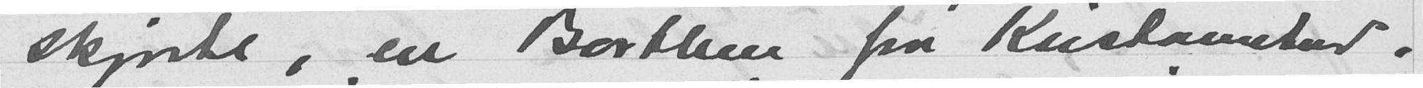skjorte, en Borthen fra Kristiansund.
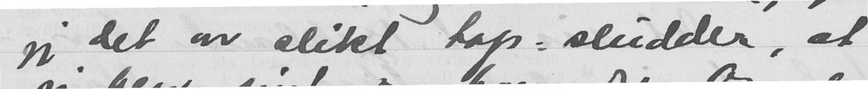jeg det var slikt tap: sludder, at
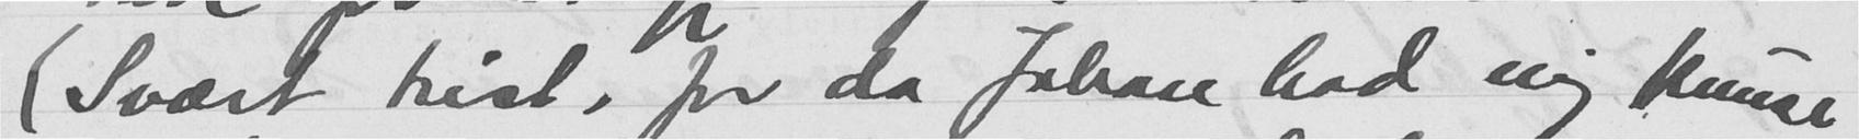(Svært trist, for da Johan bad mig knuse
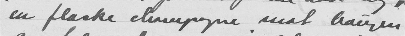en flaske champagne mot baugen
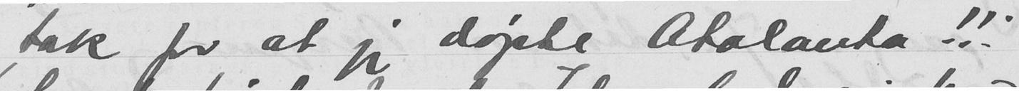tak for at jeg døpte Atalanta!!!
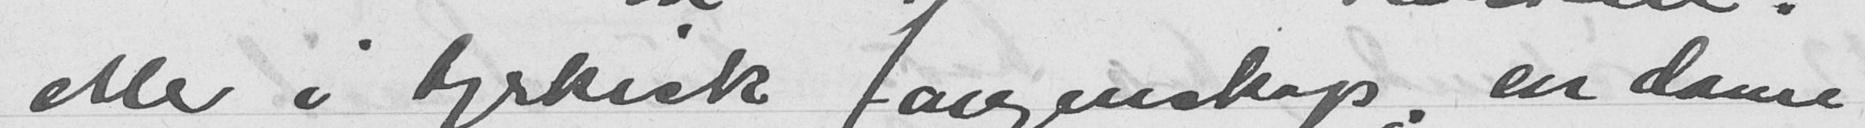eller i tyrkisk fangenskap, en dame
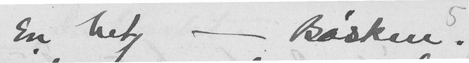En het - Bøsken.
